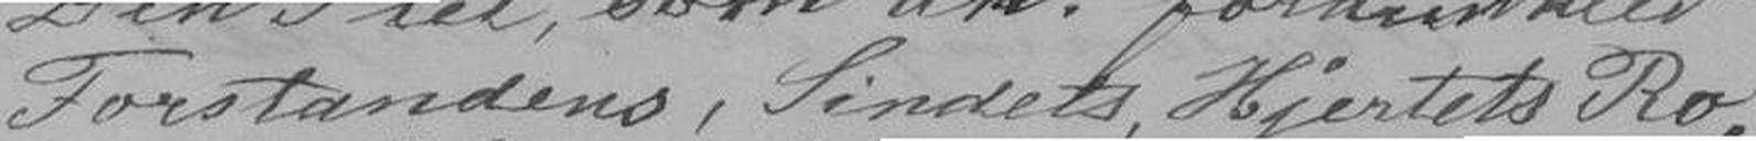Forstandens, Sindets Hjertets Ro,
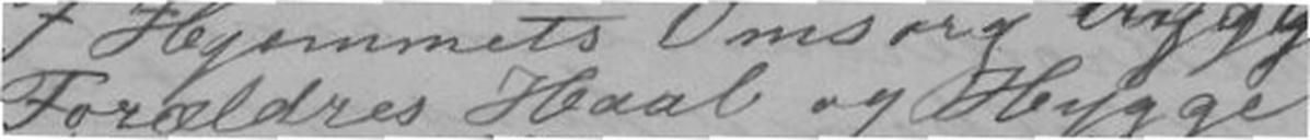Forældres Haab og Hygge
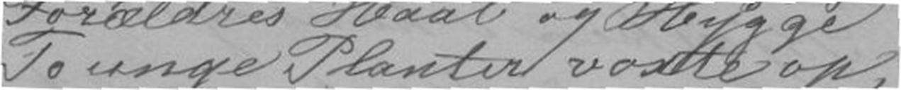To unge Planter voxte op,
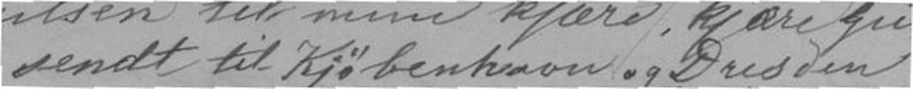sendt til Kjøbenhavn og Dresden
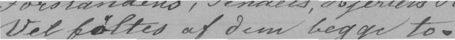Vel føltes af dem begge to.
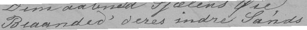Beaanded deres indre Sands
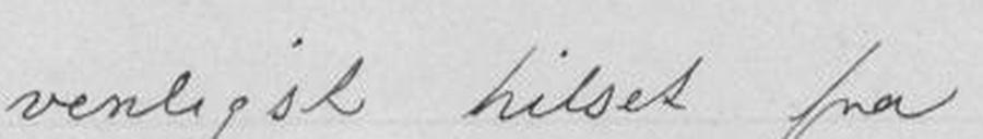venligst hilset fra
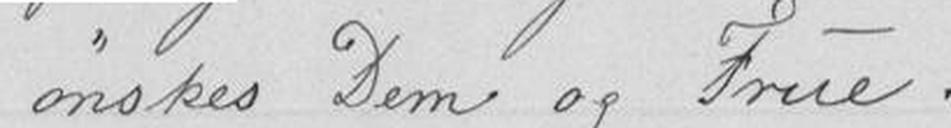ønskes Dem og Frue.
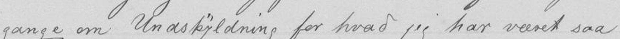gange om Undskyldning for hvad jeg har været saa
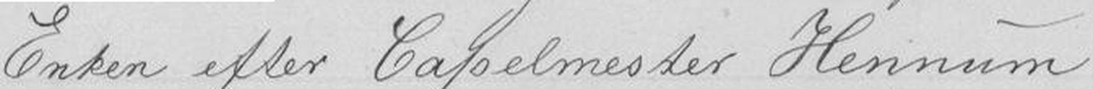Enken efter Capelmester Hennum
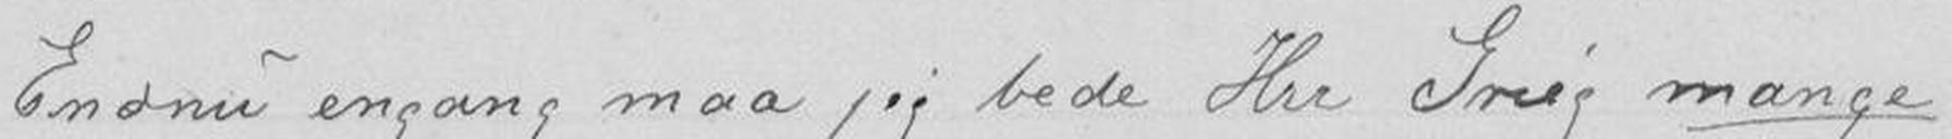Endnu engang maa jeg bede Hrr Grieg mange
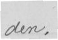den.
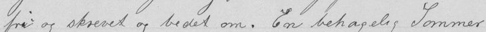fri og skrevet og bedet om. En behagelig Sommer
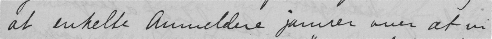at enkelte anmeldere jamrer over at vi
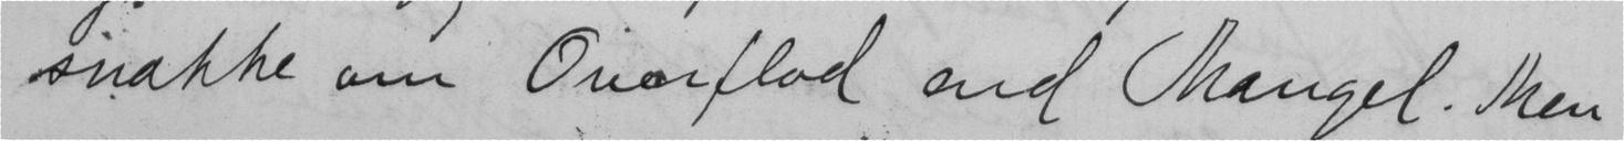snakke om Overflod end Mangel. Men
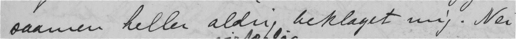saamen heller aldrig beklaget mig. Nei
